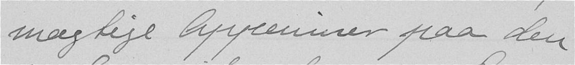mægtige appenniner paa den
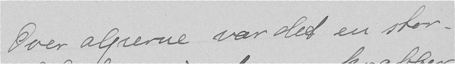Over alperne var det en stor-
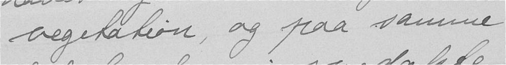vegetation, og paa samme
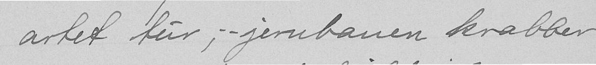- artet tur, - jernbanen krabber
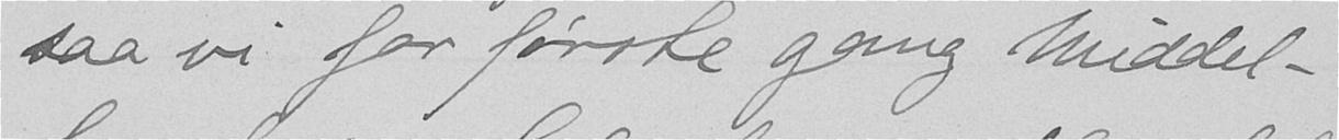saa vi for første gang Middel-
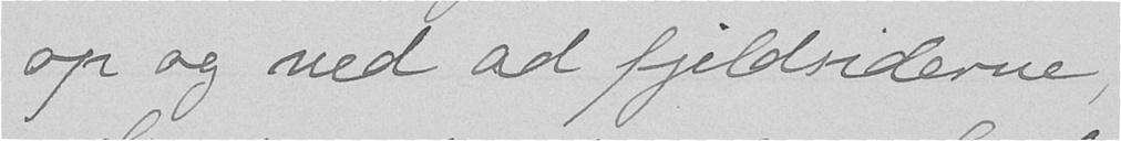op og ned ad fjeldsiderne
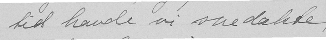tid havde vi snedækte,
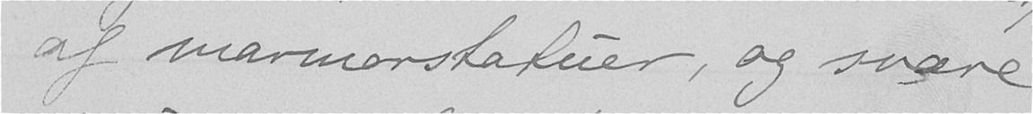af marmorstatuer, og svære
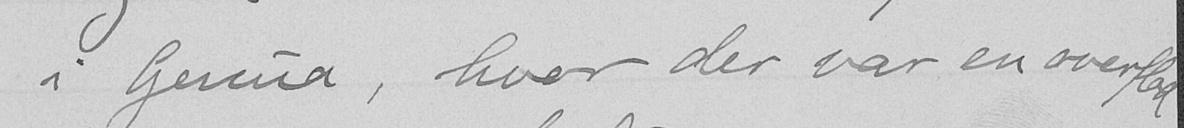i Genua, hvor der var en overflod
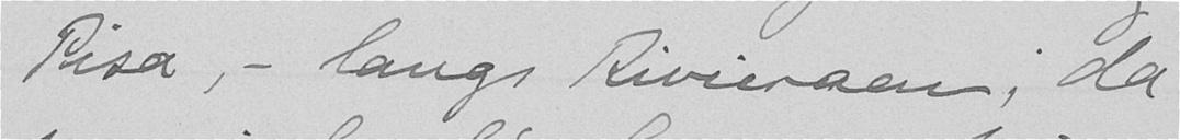Pisa, - langs Rivieraen, da
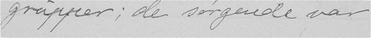grupper, de sørgende var
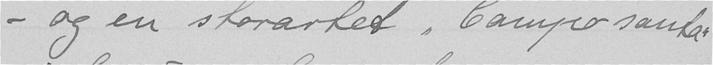- og en storartet, Campo santa
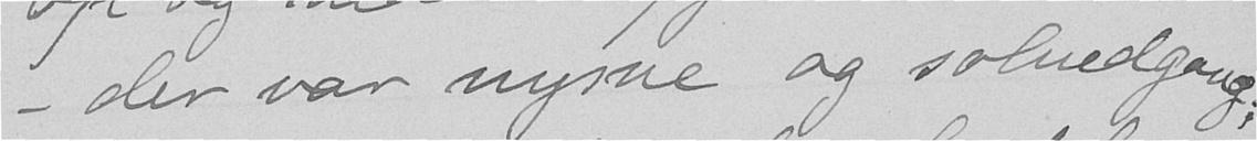- der var nysne og soldgang
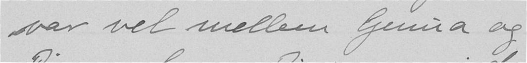var vel mellem Genua og
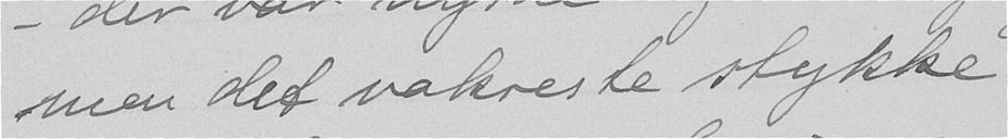men det vakreste stykke
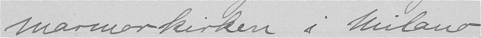marmorkirken i Milano
